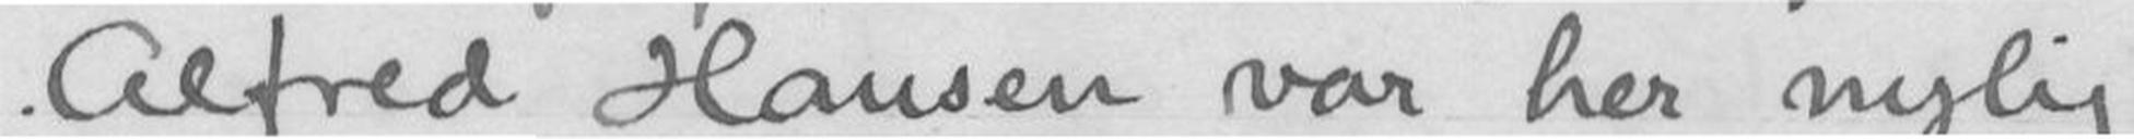Alfred Hansen var her nylig.
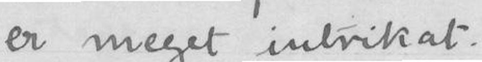er meget intrikat.
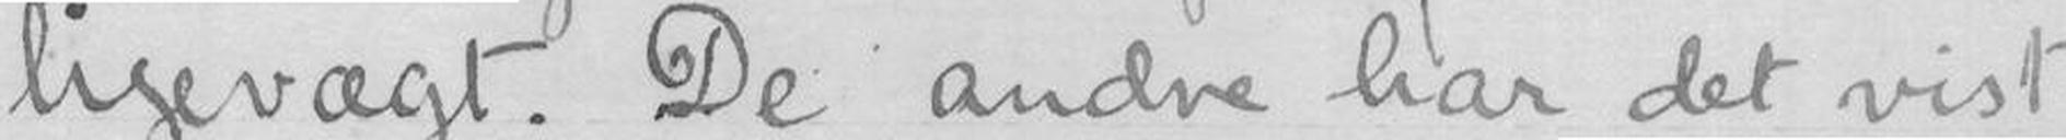ligevægt. De andre har det vist
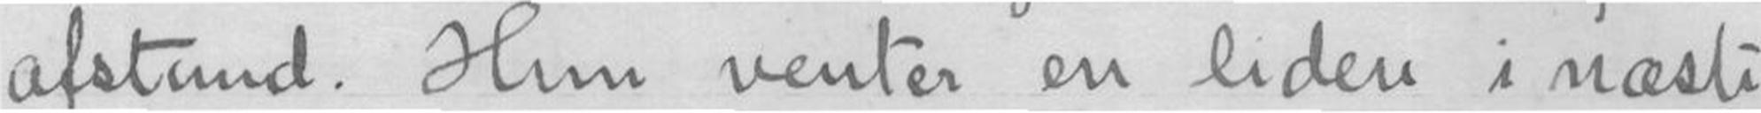afstand. Hun venter en liden i næste
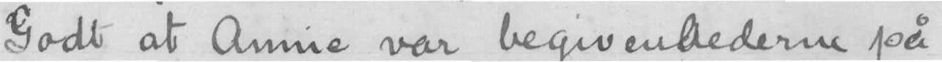Godt at Annie var begivenhederne på
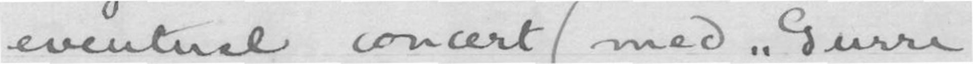eventuel concert (med Gurre
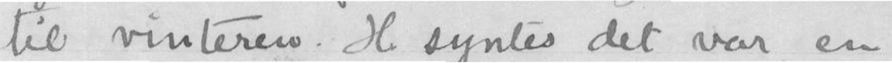til vinteren. H syntes det var en
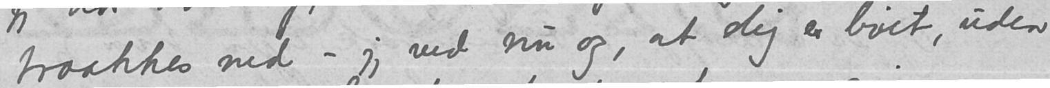traakkes ned - jeg ved nu og, at dig er livet, uden
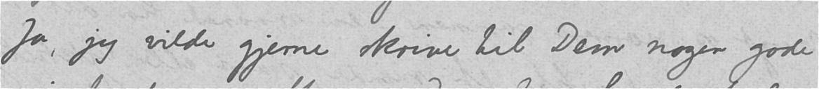Ja, jeg vilde gjerne skrive til Dem nogen gode
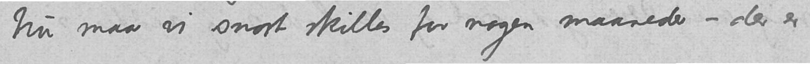Nu maa vi snart skilles for nogen maaneder – der er
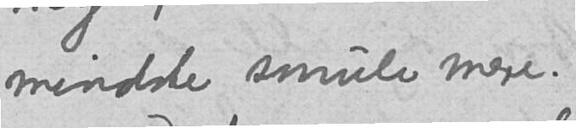mindste smule mere.
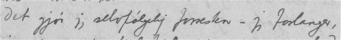Det gjør jeg selvfølgelig forresten – jeg forlanger,
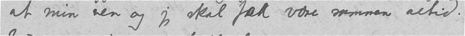at min ven og jeg skal faa være sammen altid.
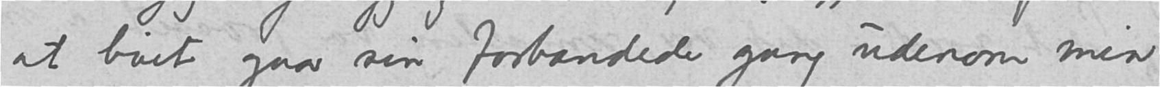at livet gaar sin forbandede gang udenom min
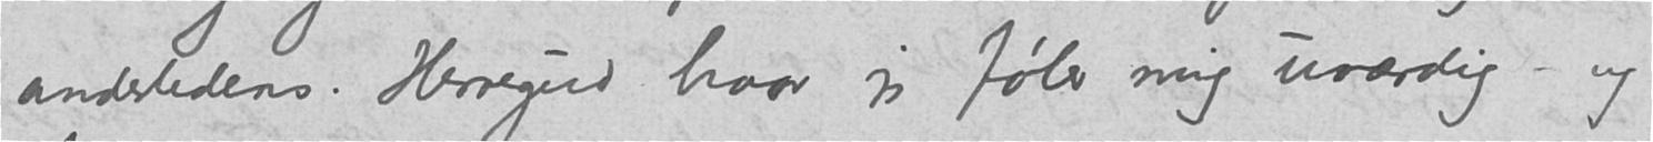anderledens. Herregud hvor jeg føler mig uværdig - og
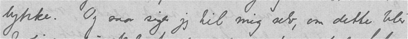lykke. Og saa siger jeg til mig selv, om dette blir
